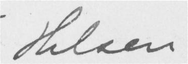Hilsen
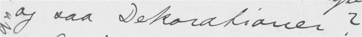og saa Dekorationer?
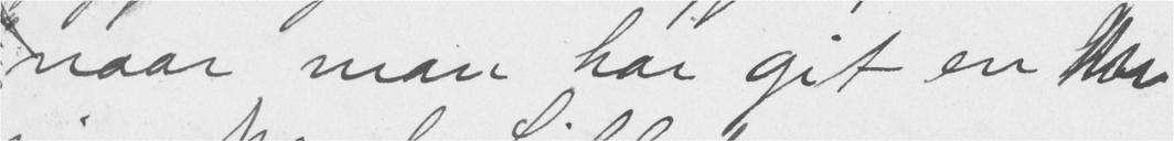naar man har git en
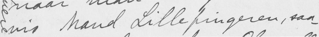vis Mand Lillefingeren, saa-
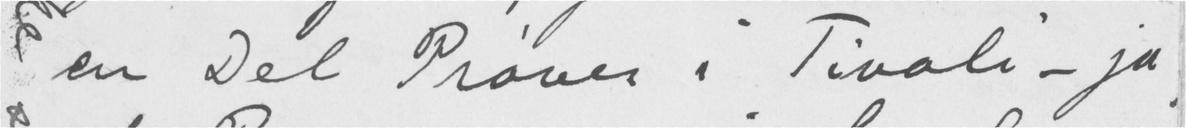en Del Prøver i Tivoli — ja
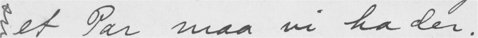et Par maa vi ha der.
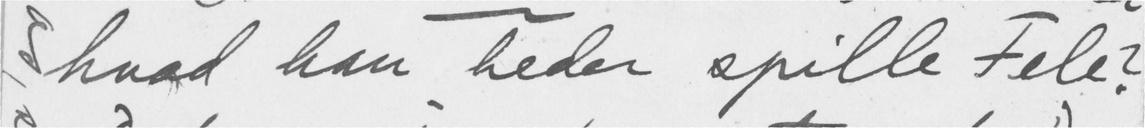hvad han heder spille Fele?
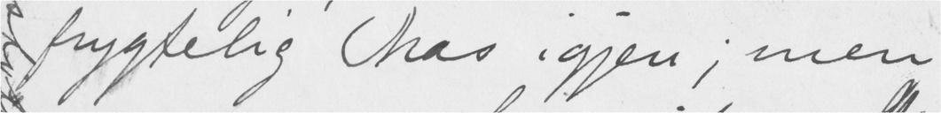frygtelig Mas igjen; men
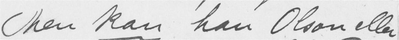Men kan han Olson eller
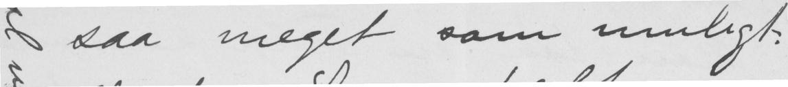saa meget som muligt.
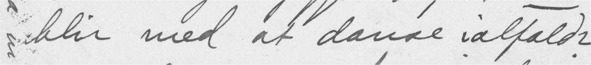blir med at danse ialfald?
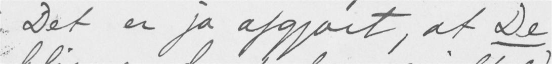Det er jo afgjort, af De
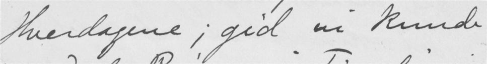Hverdagene; gid vi kunde
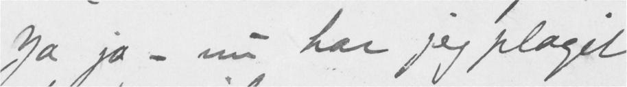Ja ja - nu har jeg plaget
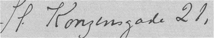St. Kongensgade 21,
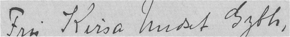Fru Kirsa Undset Gyth,
